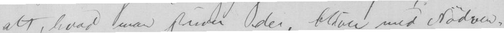alt, hvad man skriver der, bliver med Nødven-
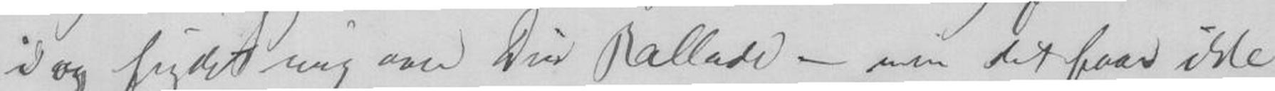i og frydet mig over Din Ballade - men det faar ikke
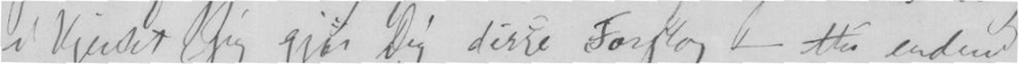i Hjertet jeg gjør Dig disse Forslag - thi endnu
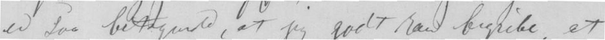er saa betagende, at jeg godt kan begribe, at
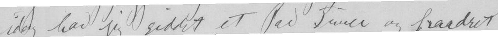idag har jeg siddet et Par Timer og fraadset
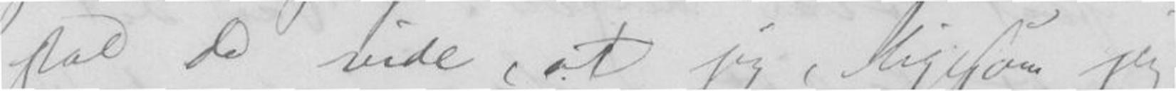skal Du vide, at jeg, ligesom jeg
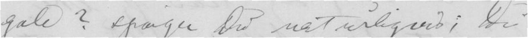gale? spørger Du naturligvis; Da
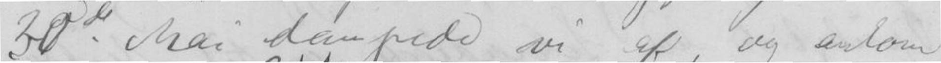30de Mai dampede vi af, og ankom
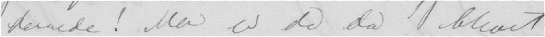dernede! Men er de da blivet
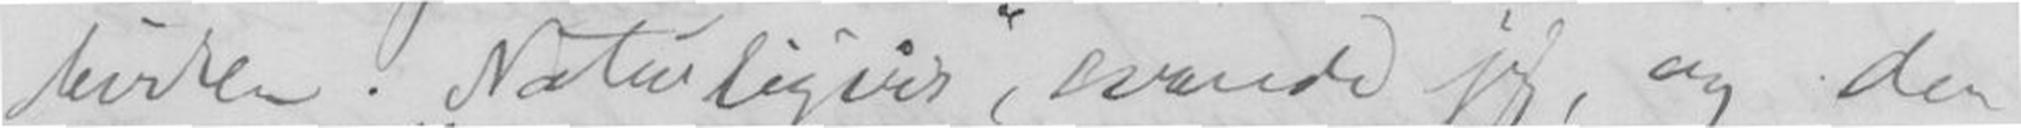kirken. Naturligvis, svarede jeg, og den
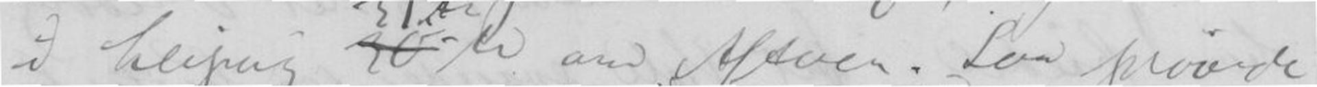i Leipzig 30te om Aftnen. Saa prøvde
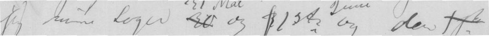jeg mine Sager 30 Mai og 1ste Juni og den 1ste
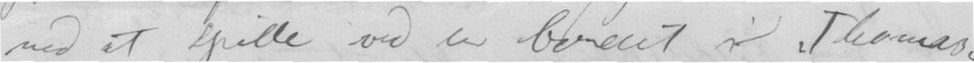med at spille ved en Concert i "Thomas-
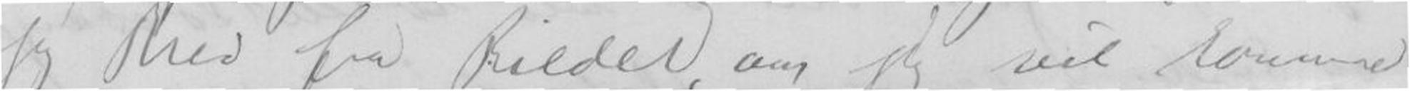jeg Brev fra Rieder, om jeg vil komme
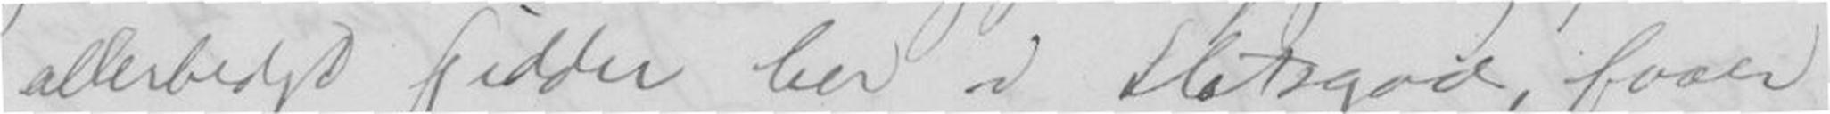allerbedst sidder her i Slotsgade, faaer
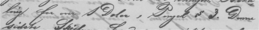ling for over 1 Daler, Pengel §3. Denne
